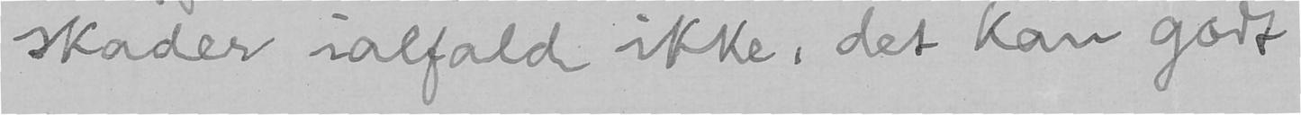skader ialfald ikke, det kan godt
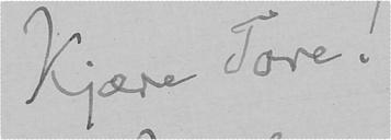Kjære Tore!
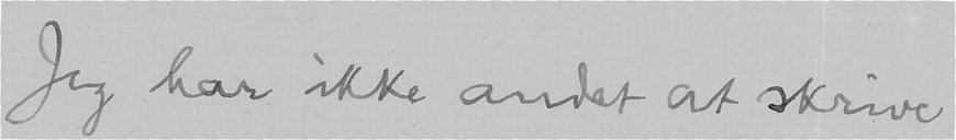Jeg har ikke andet at skrive
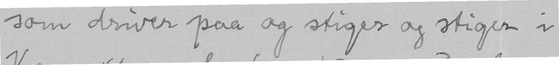som driver paa og stiger og stiger i
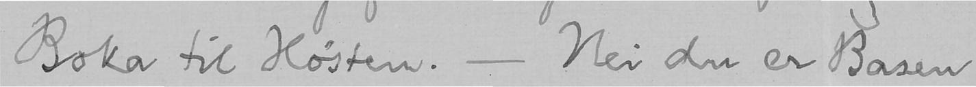Boka til Høsten. - Nei du er Basen
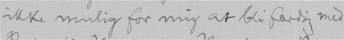ikke mulig for mig at bli færdig med
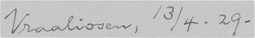Vraaliosen, 13/4. 29.
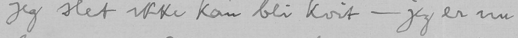jeg slet ikke kan bli kvit - jeg er nu
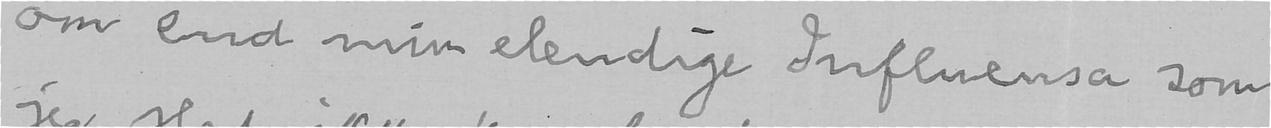om end min elendige Influensa som
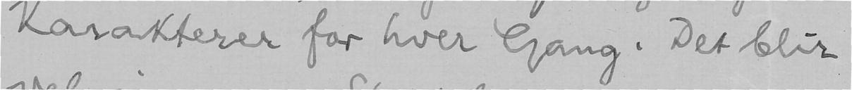Karakterer for hver Gang. Det blir
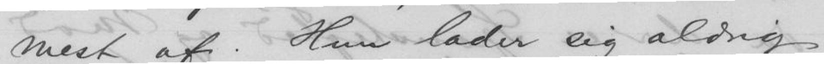mest af. Hun lader sig aldrig
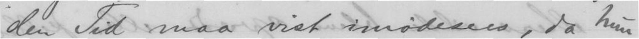den Tid maa vist imødesees, da hun
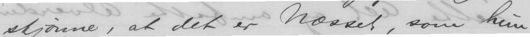skjønne, at det er Næsset, som hun
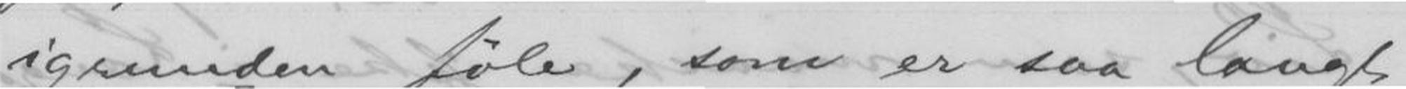igrunden føle, som er saa langt
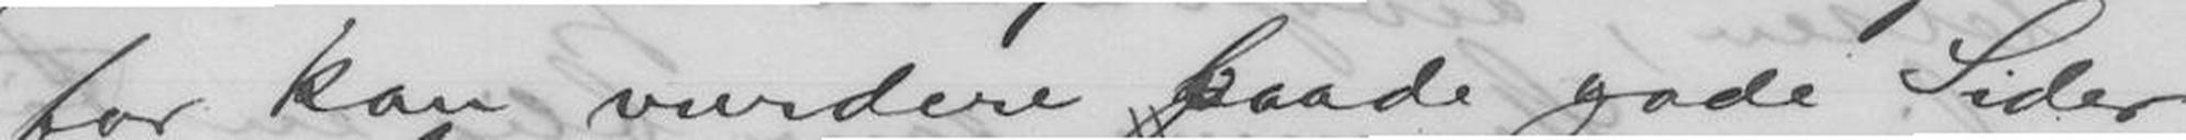for kan vurdere baade gode Sider
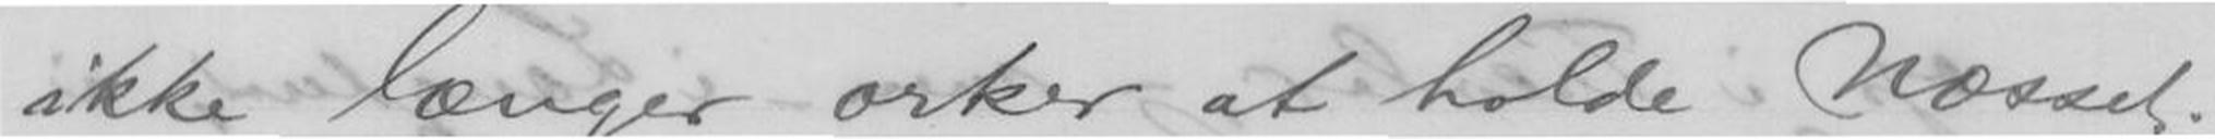ikke længer orker at holde Næsset.
